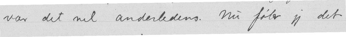var det vel anderledens. Nu føler jeg det
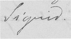Sigrid.
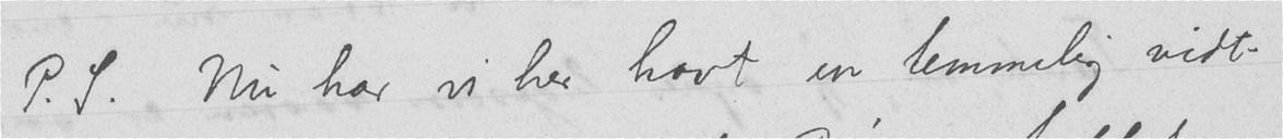P.S. Nu har vi her en temmelig vidt-
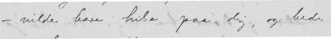- vilde bare hilse paa dig, og bede
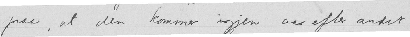paa, at den kommer igjen aar efter andet
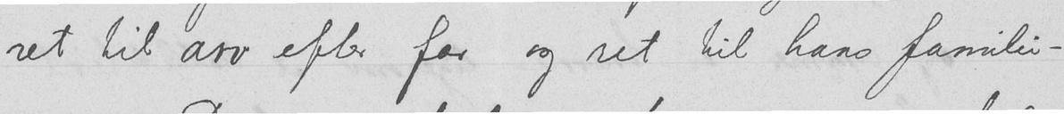ret til arv efter far og ret til hans familie-
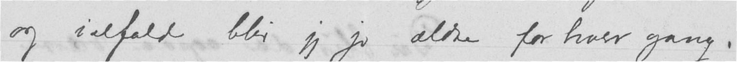og ialfald blir jeg jo ældre for hver gang.
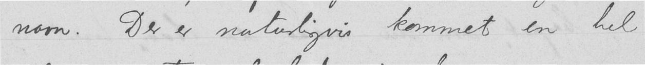navn. Der er naturligvis kommet en hel
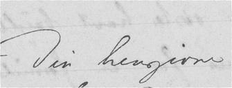Din hengivne
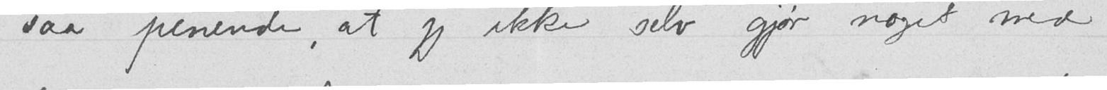saa pinende, at jeg ikke selv gjør noget med
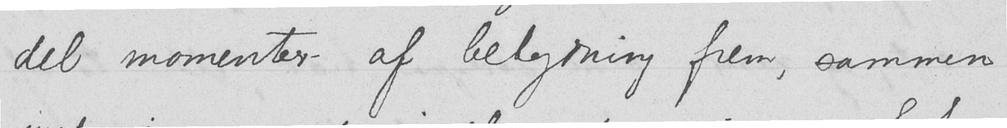del momenter af betydning frem, sammen
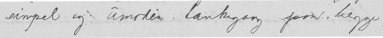simpel og umoden tankegang paa begge
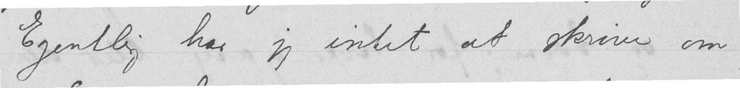Egentlig har jeg intet at skrive om
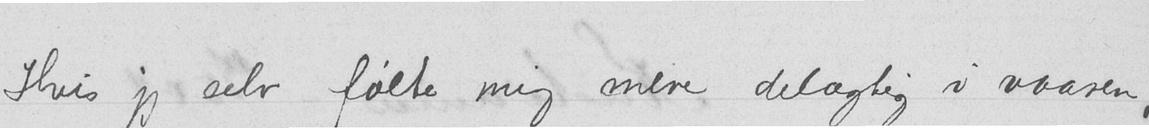Hvis jeg selv følte mig mere delagtig i vaaren,
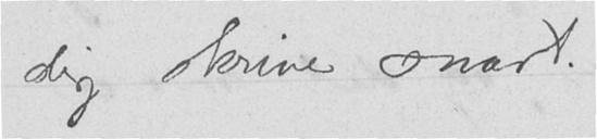dig skrive snart.
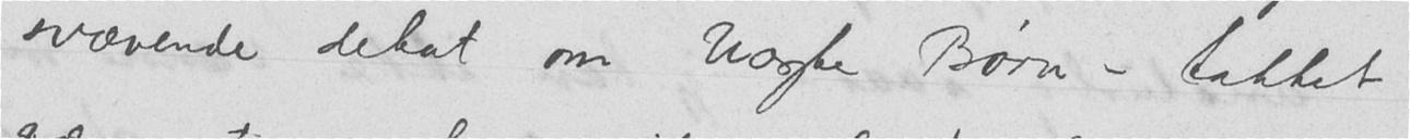svævende debat om Uægte Børn - takket
